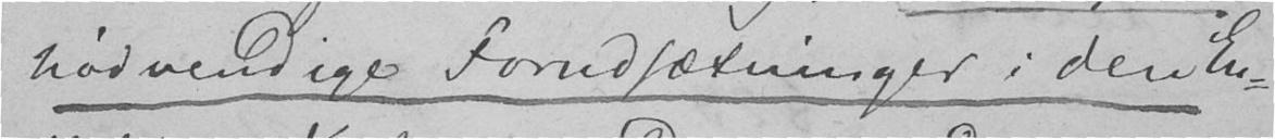nødvendige Forudsætninger i den En-
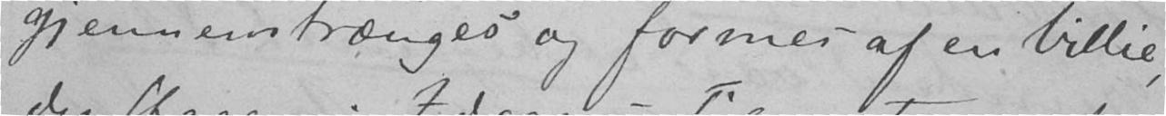gjennemtrænges og formes af en Villie,
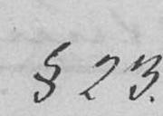§ 23.
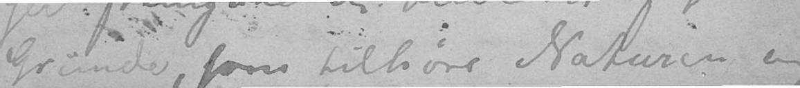Grunde, som tilhøre Naturen og
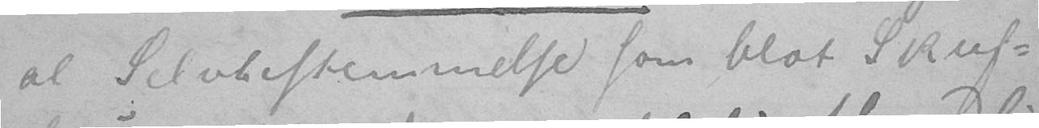al Selvbestemmelse som blot Skuf-
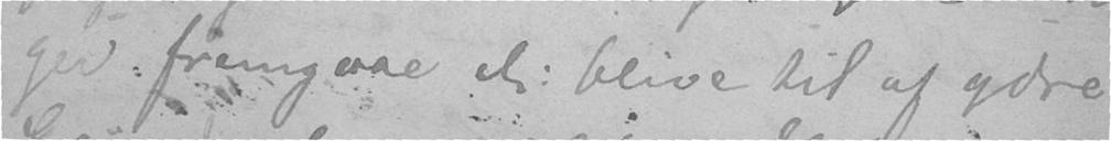ger fremgaae el: blive til af ydre
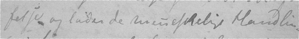felse og lader de menneskelige Handlin-
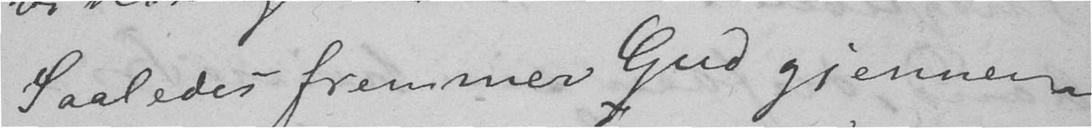Saaledes fremmer Gud gjennem
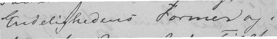Endelighedens Former og
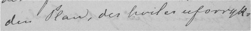den Plan, der hviler uforryk-
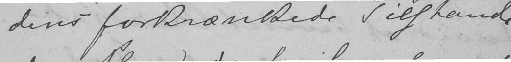dens forkrænkede Tilstand
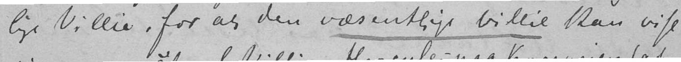lige Villie, for at den væsentlige Villie kan vise
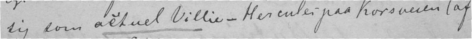sig som actuel Villie – Herunder paa Korsveien (af
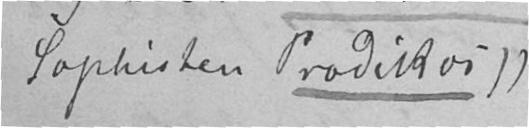Sophisten Prodikos))
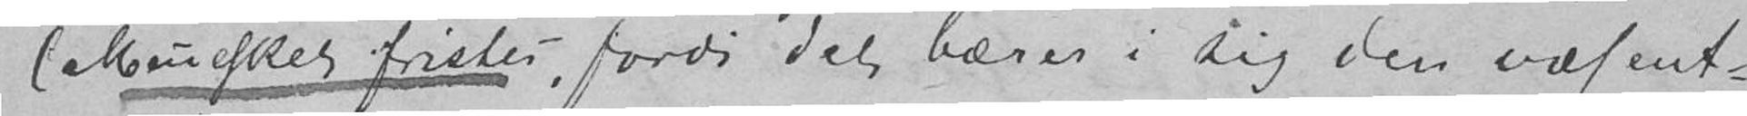(Mennesket fristes, fordi det bærer i sig den væsent-
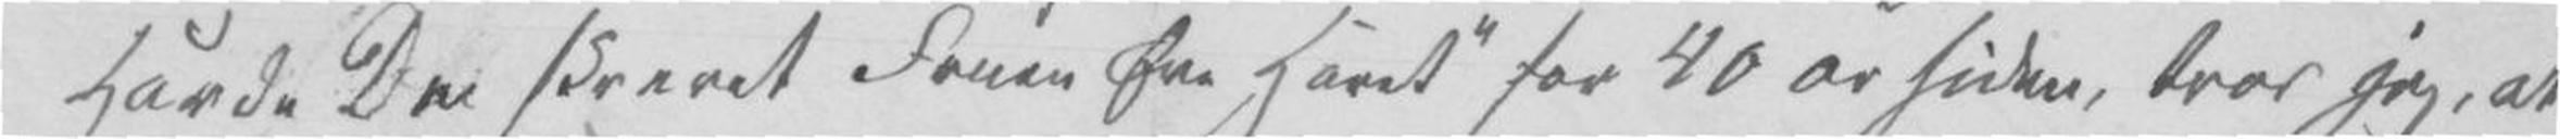Havde De skrevet [«]Fruen fra Havet» for 40 år siden, tror jeg, at
